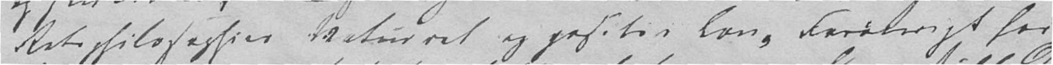Retsphilosophies Naturret og positiv Lov. Forøvrigt har
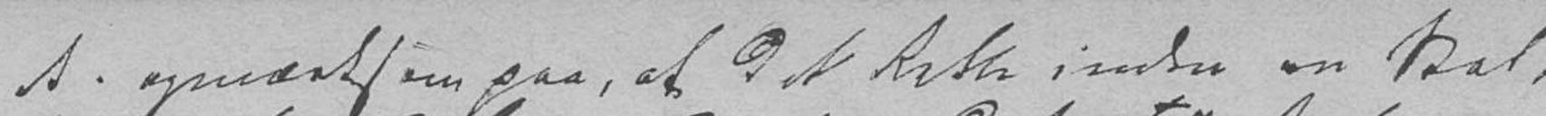A. opmærksom paa, at det Rette inden en Stat
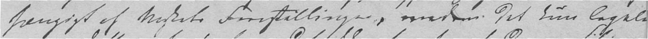hængigt af Mskets Forestillinger, medens det kun legale
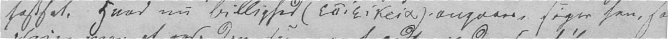fastsat. Hvad nu Billighed (gresk) angaaer, siger han, saa
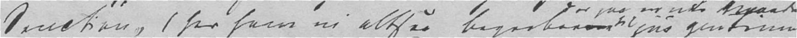Sanction (her have vi altsaa Begreber til jus gentium
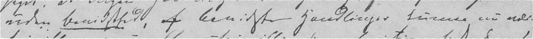uden Bevidsthed, af bevidste Handlinger kunne nu være
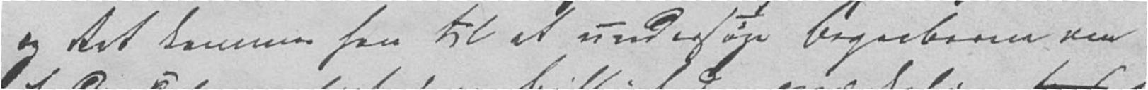og Ret kommer han til at undersøge Begreberne
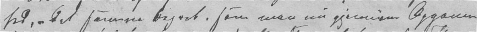hed. Det samme Begreb, som man nu gjennem Opgaven
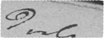deels er
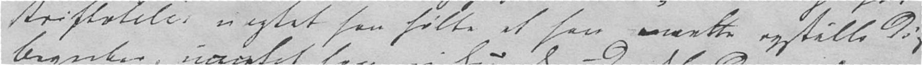Aristoteles uagtet han følte at han maatte opstille disse
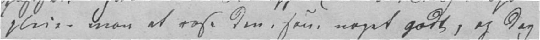pleier man at rose den, som noget godt, og dog
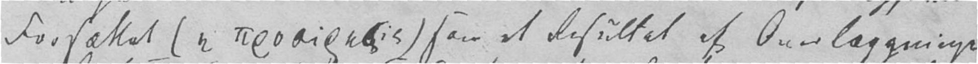Forsættet (gresk) som et Resultat af Overlæggninger.
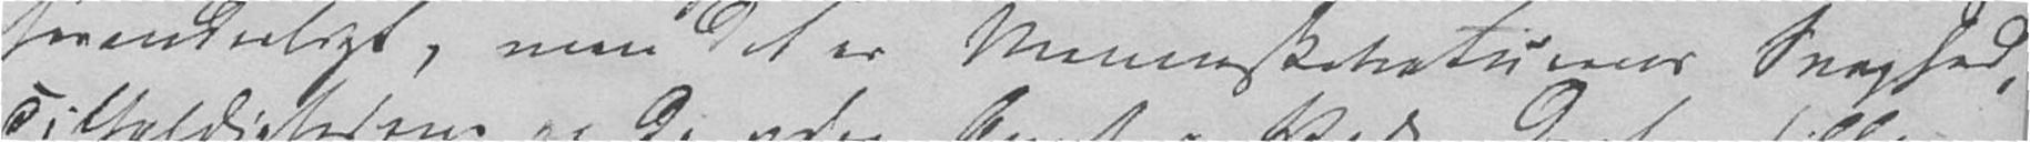foranderligt, men det er Menneskenaturens Svaghed,
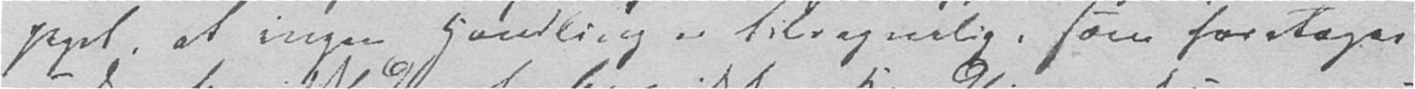peget, at ingen Handling er tilregnelig, som foretages
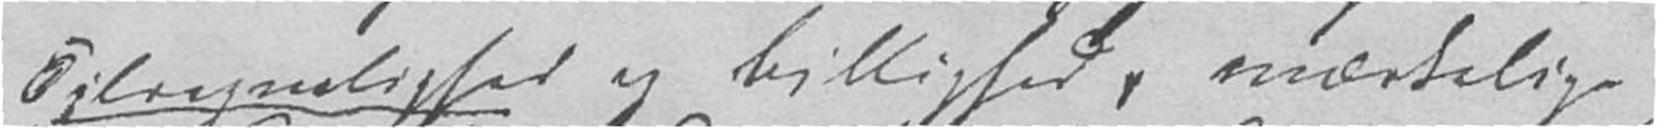Tilregnelighed og Billighed, mærkelige
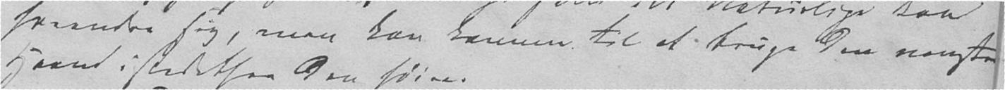forandre sig, man kan komme til at bruge den venstre
